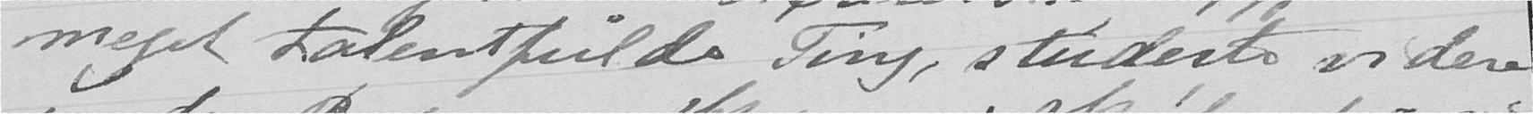meget talentfulde Ting, studerte videre
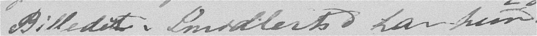Billeder. Imidlertid har hun
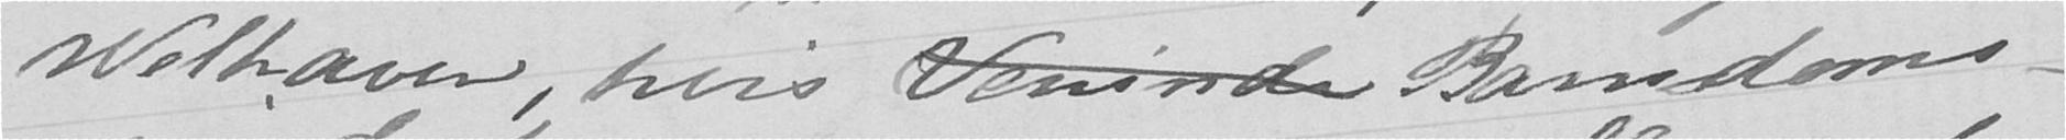Welhaven, hvis Veninde Barndoms-
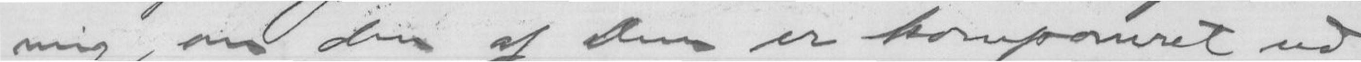mig, om den af Dem er komponeret ud
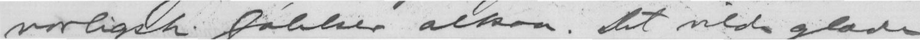vorligste følelser altsaa. Det vilde glæde
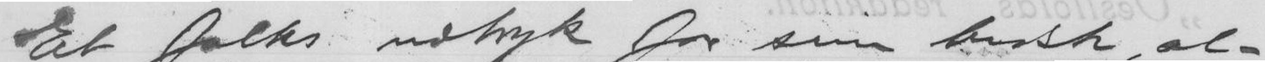Et folks udtryk for sin bedste, al-
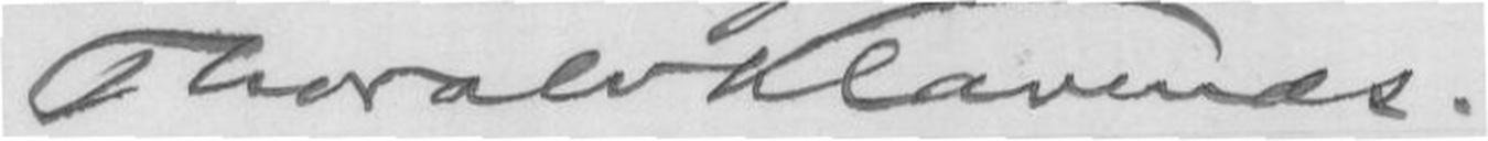Thorvald Klavenæs.
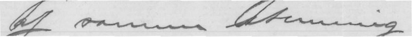af samme Stemning.
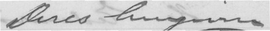Deres hengivne
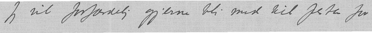Jeg vil forfærdelig gjerne bli med til festen for
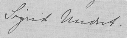Sigrid Undset.
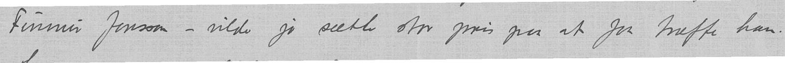Finnur Jonsson – vilde jo sætte stor pris paa at faa træffe ham.
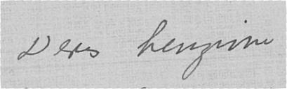Deres hengivne
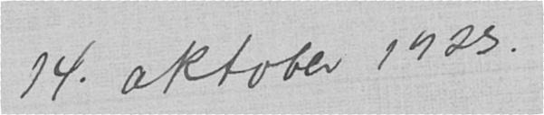14. oktober 1923.
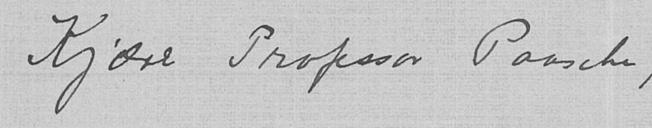Kjære Professor Paasche,
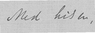Med hilsen,
